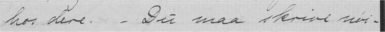hos dere. - Du maa skrive nøi-
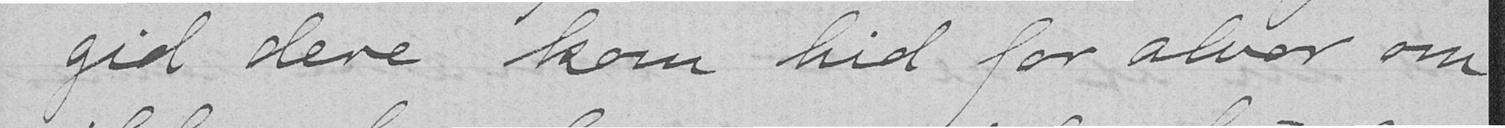gid dere kom hid for alvor om
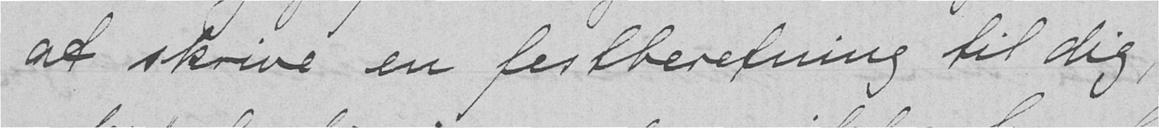at skrive en festberetning til dig,
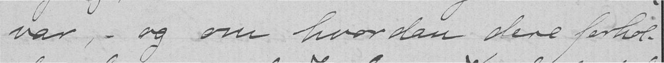var, - og om hvordan dere forhol-
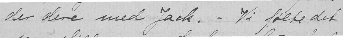der dere med Jack. - Vi følte det
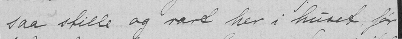saa stille og rart her i huset, før
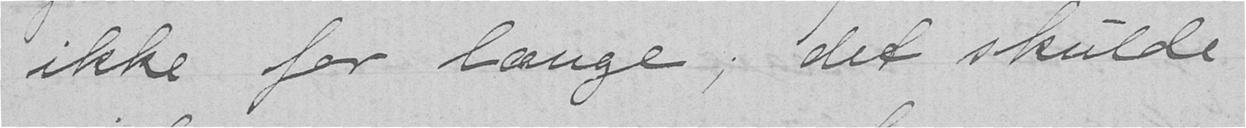ikke for længe; det skulde
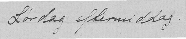Lørdag eftermiddag.
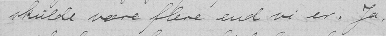skulde være flere end vi er. Ja,
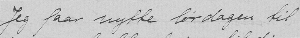Jeg faar nytte lørdagen til
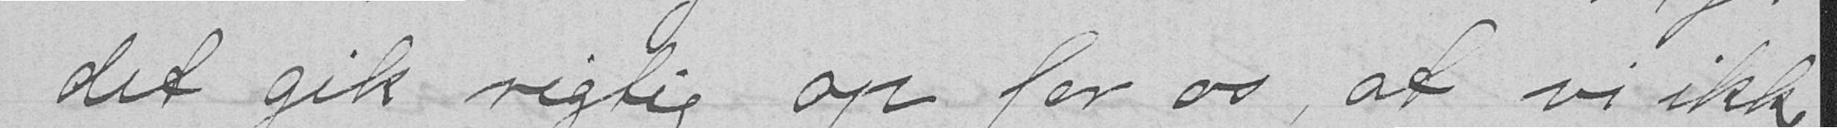det gik rigtig op for os, at vi ikke
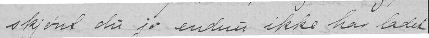skjønt du jo endnu ikke har ladet
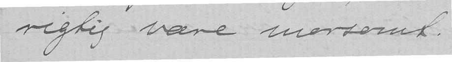rigtig være morsomt.
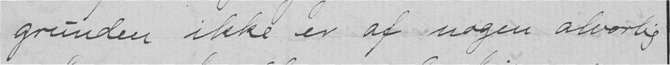grunden ikke er af nogen alvorlig
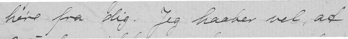høre fra dig. Jeg haaber vel, at
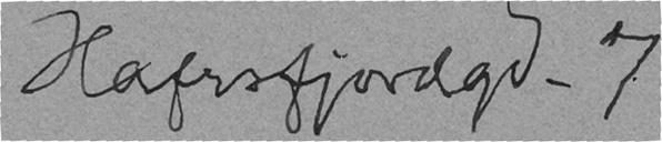Hafrsfjordgd. 7
